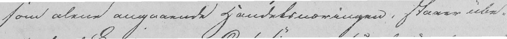som alene angaaende Handelsnæringen, staaer ube-
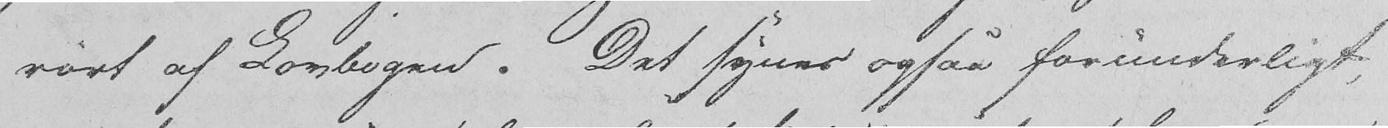rørt af Lovbogen. Det synes ogsaa forunderligt,
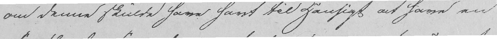om denne skulde have havt til Hensigt at have en
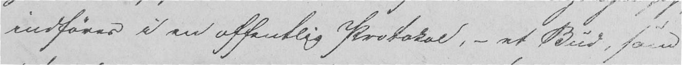indføres i en offentlig Protokol, – et Bud, som
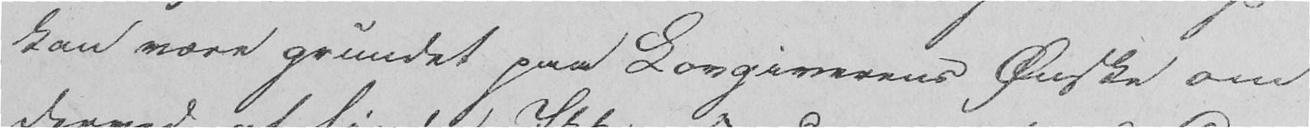kan være grundet paa Lovgiverens Ønske om
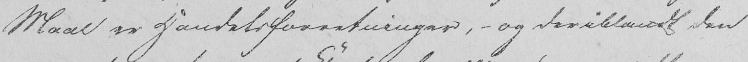Maal er Handelsforretninger, - og deriblandt den
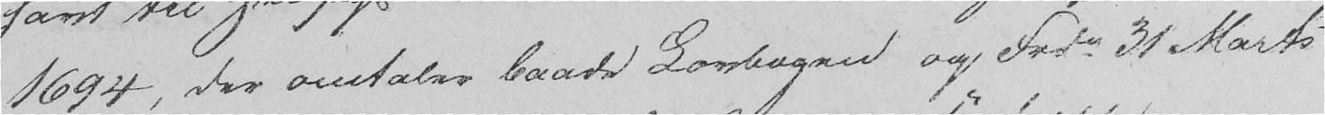1694, der omtaler baade Lovbogen og Frdn 31 Marts
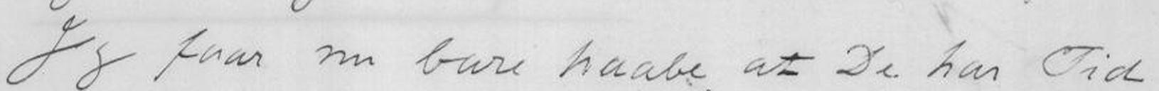Jeg faar nu bare haabe, at De har Tid
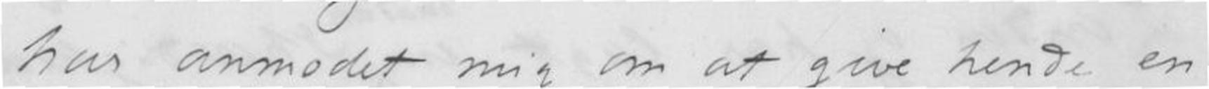har anmodet mig om at give hende en
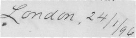London, 24/1/96
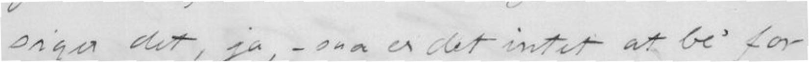sige det, ja, - saa er det intet at bi for.
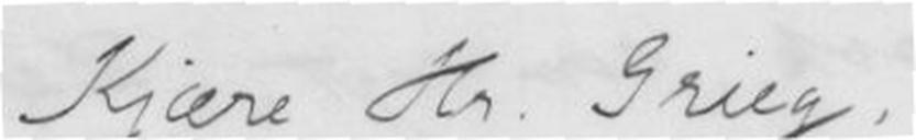Kjære Hr. Grieg.
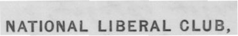NATIONAL LIBERAL CLUB.
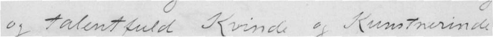og talentfuld Kvinde og Kunstnerinde
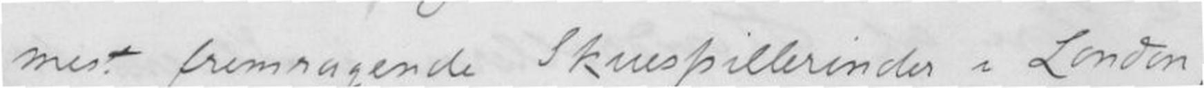mest fremragende Skuespillerinder i London,
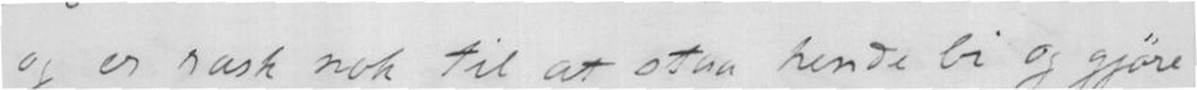og er rask nok til at staa hende bi og gjøre
